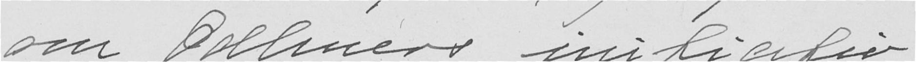om Odhners initiativ
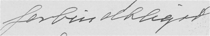forbindtligst
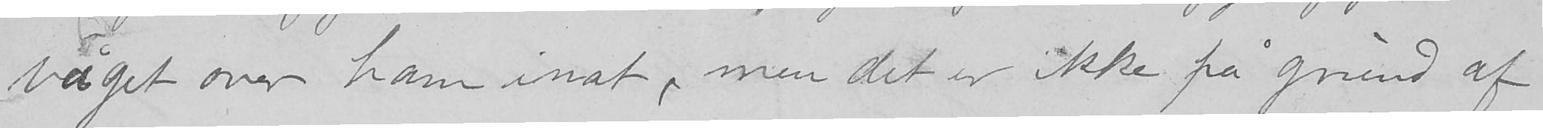våget over ham inat, men det er ikke på grund af
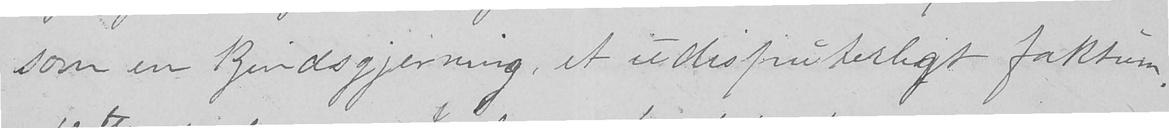som en kjendsgjerning, et udisputerligt faktum.
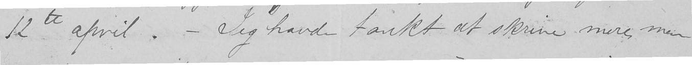12te april. - Jeg havde tænkt at skrive mere, men
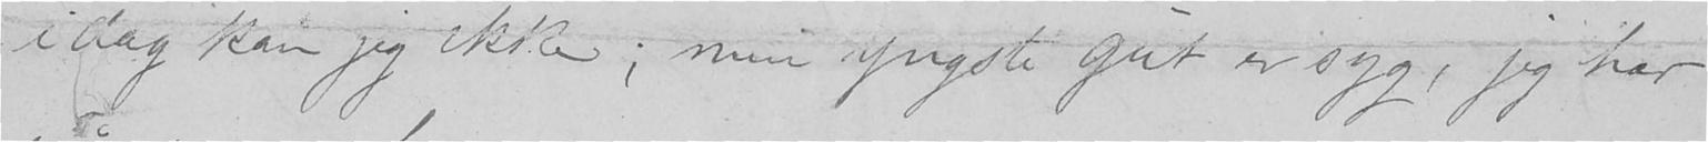i dag kan jeg ikke; min yngste gut er syg, jeg har
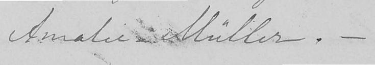Amalie Müller. -
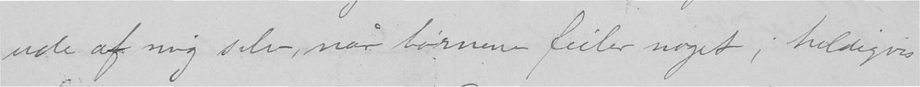ude af mig selv, når børnene feiler noget; heldigvis
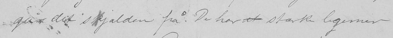går det skjælden på. De har et stærke legemer
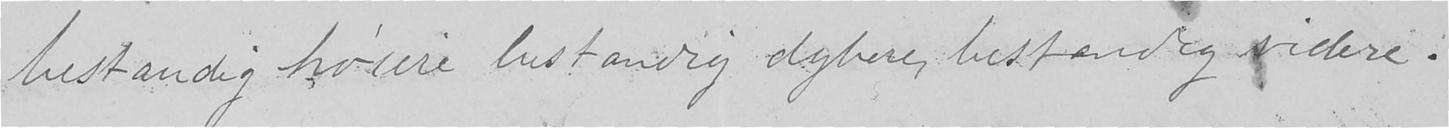bestandig høiere bestandig dybere, bestandig videre.
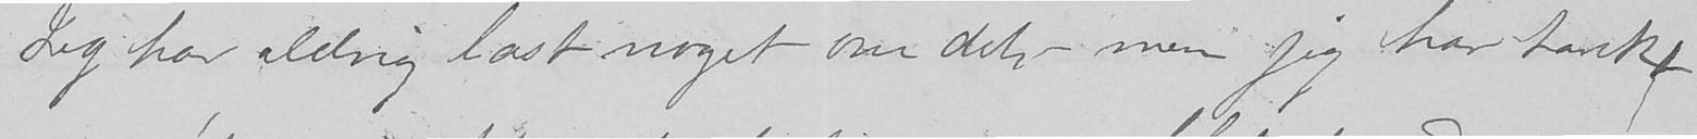Jeg har aldrig læst noget om det,- men jeg har tænkt
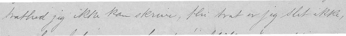træthed jeg ikke kan skrive, thi træt er jeg slet ikke,
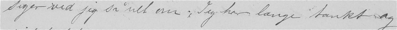siger ved jeg så vel om. Jeg har længe tænkt og
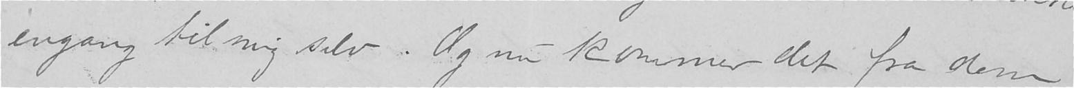engang til mig selv. Og nu kommer det fra dem
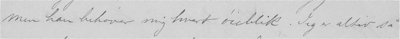men han behøver mig hvert øieblik. Jeg er altid så
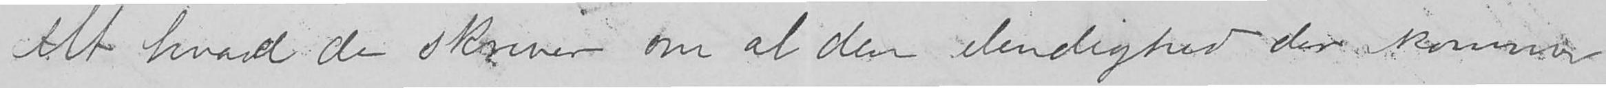Alt hvad de skriver om al den elendighed der kommer
